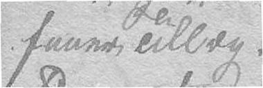faaer Tillæg.
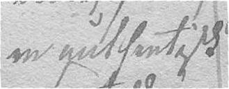en authentisk
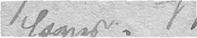af Loven ey
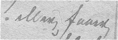eller faaer
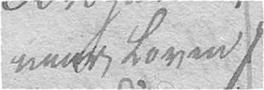naar Loven
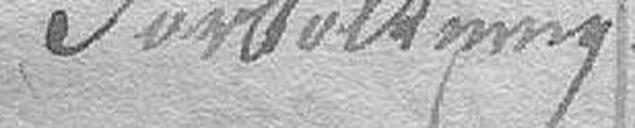Fortolkning.
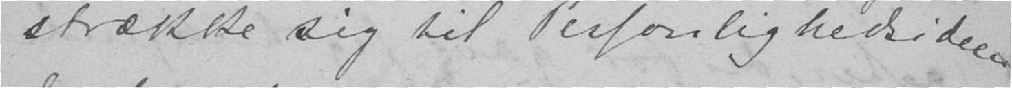strække sig til Personlighedsideen
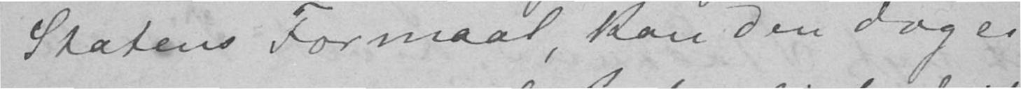Statens Formaal, kan den dog ei
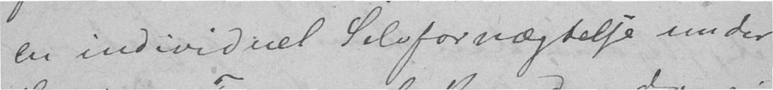en individuel Selvfornægtelse under
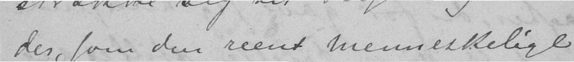der, som den reent menneskelige
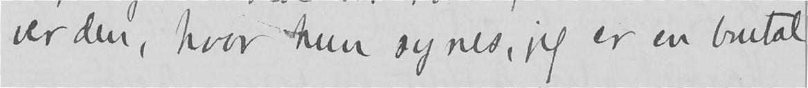verden, hvor hun synes, jeg er en brutal
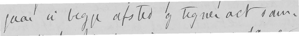gaar vi begge afsted og tegner act sam-
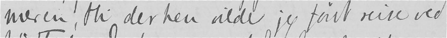meren, thi derhen vilde jeg først reise ved
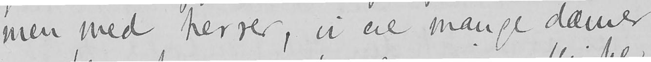men med herrer, vi ere mange damer
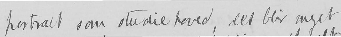portrait som studiehoved, det blir meget
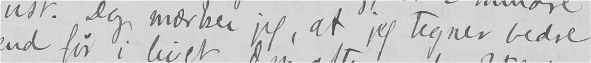vist. Dog mærker jeg, at jeg tegner bedre
